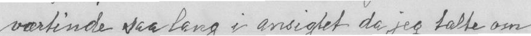værtinde saa lang i ansigtet da jeg talte om
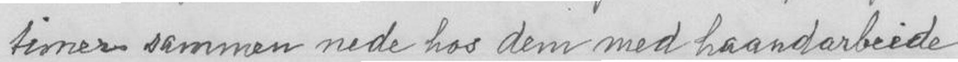timer sammen nede hos dem med haandarbeide
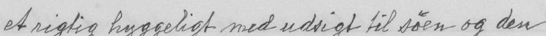et rigtig hyggeligt med udsigt til søen og den
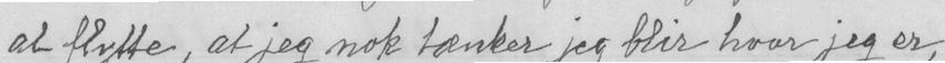at flytte, at jeg nok tænker jeg blir hvor jeg er,
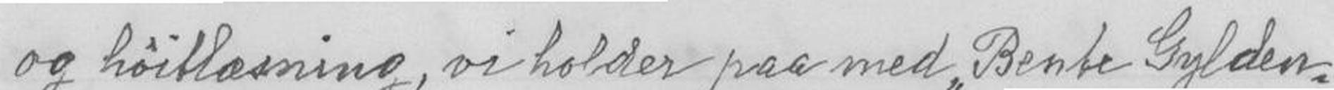og høitlæsning, vi holder paa med "Bente Gylden-
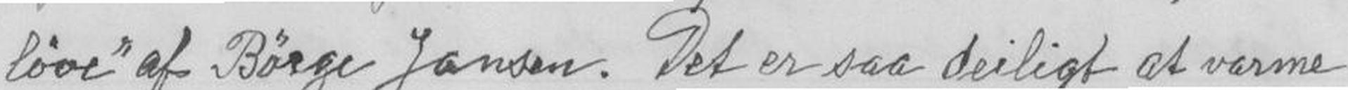løve" af Børge Janson. Det er saa deiligt at varme
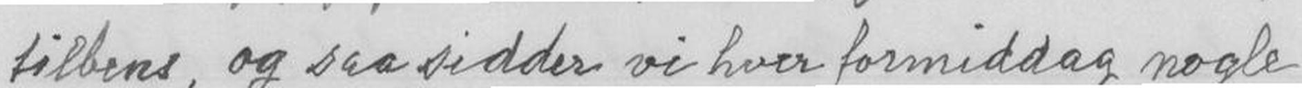tilbens, og saa sidder vi hver formiddag nogle
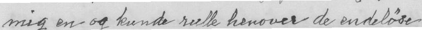mig en og kunde rulle henover de endeløse
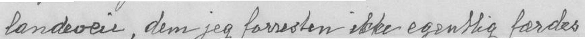landeveie, dem jeg forresten ikke egentlig færdes
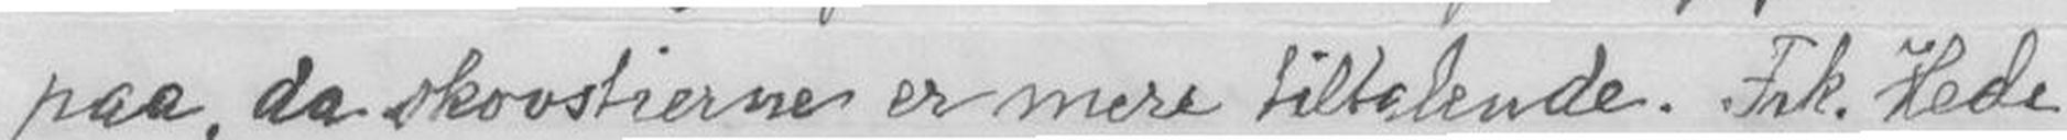paa, da skovstierne er mere tiltalende. Frk. Hede-
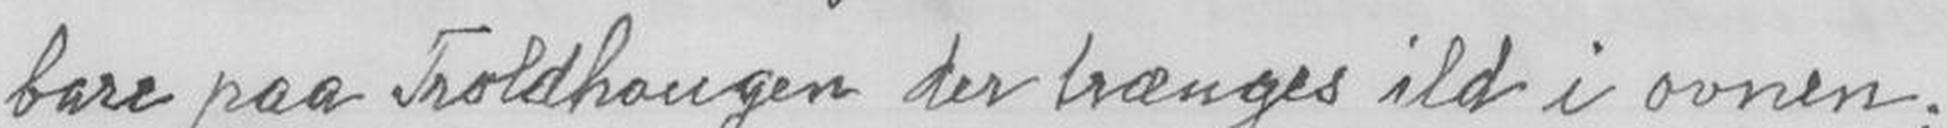bare paa Troldhaugen der trænges ild i ovnen;
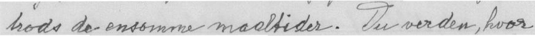de ensomme naaltider. Du verden, hvor
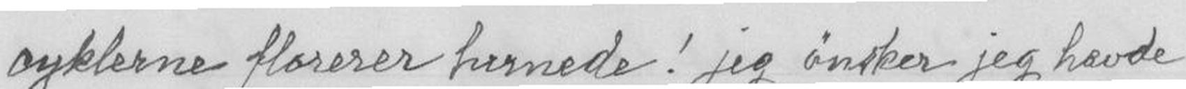syklerne florerer tunede! jeg ønsker jeg havde
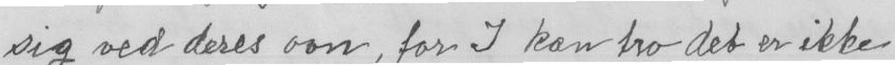sig ved deres ovn, for I kan tro det er ikke
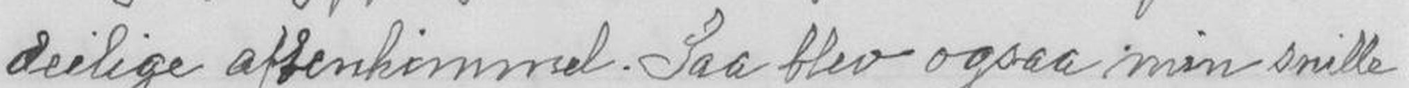deilige aftenhimmel. Saa blev ogsaa min snille
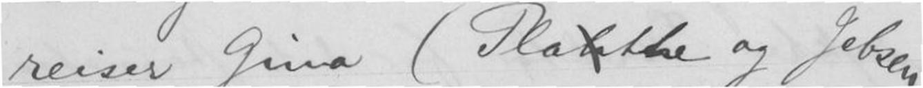reiser Gina (Plathe, og Jebsen
